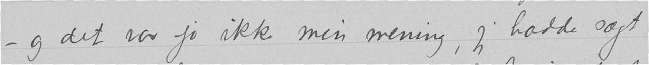– og det var jo ikke min mening, jeg hadde sagt
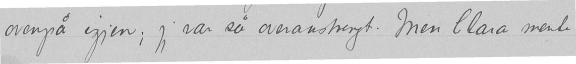ovenpå igjen; jeg var så overanstrengt. Men Clara mente
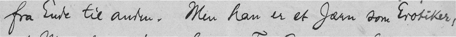fra Ende til anden. Men han er et Jærn som Erotiker,
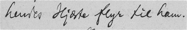hendes Hjærte flyr til ham.
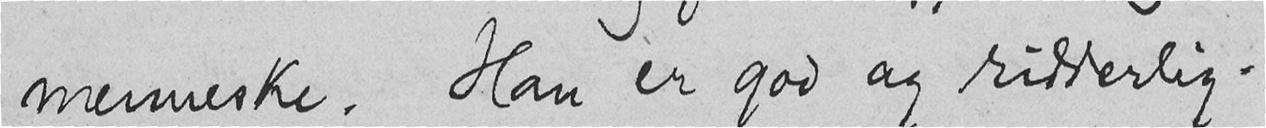menneske. Han er god og ridderlig.
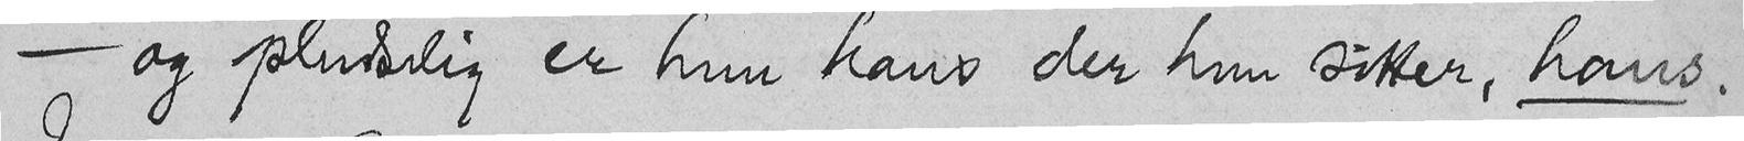- og pludselig er hun hans der hun sitter, hans.
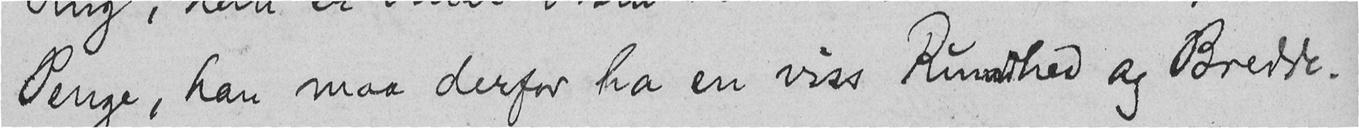Penge, han maa derfor ha en viss Rundhed og Bredde.
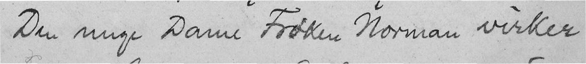Den unge Dame Frøken Norman virker
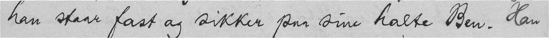han staar fast og sikker paa sine halte Ben. Han
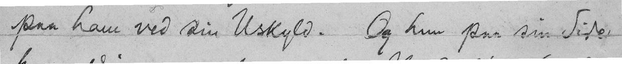paa ham ved sin Uskyld. Og hun paa sin Side
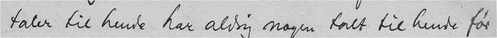taler til hende har aldrig nogen talt til hende før
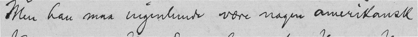Men han maa ingenlunde være nogen amerikansk
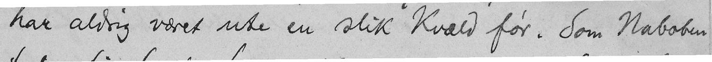har aldrig været ute en slik Kvæld før. Som Naboben
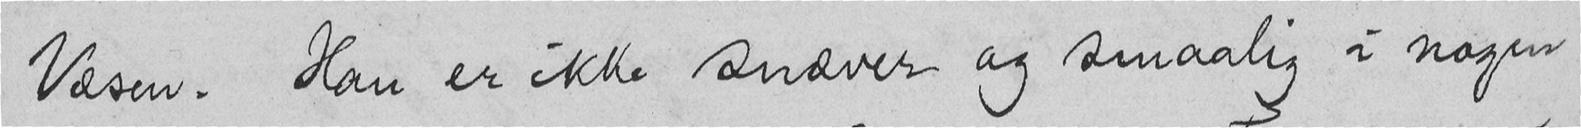Væsen. Han er ikke snæver og smaalig i nogen
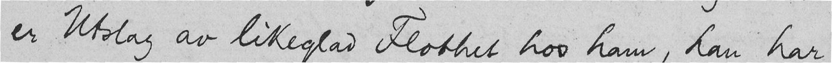er Utslag av likeglad Flothet hos ham, han har
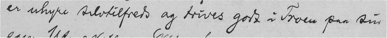er uhyre selvtilfreds og trives godt i Troen paa sin
